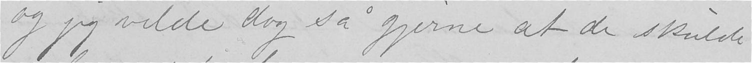og jeg vilde dog så gjerne at de skulde
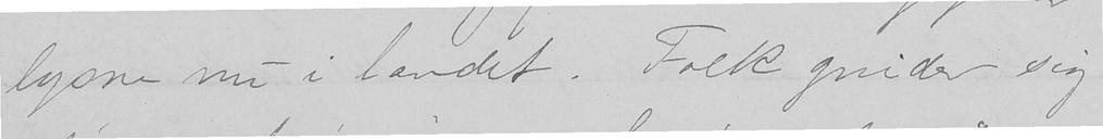lysne nu i landet. Folk gnider sig
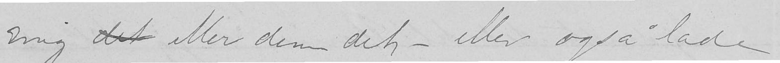mig det eller dem det, - eller også lade
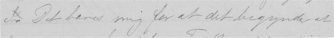Tro Det bæres mig for at det begynder at
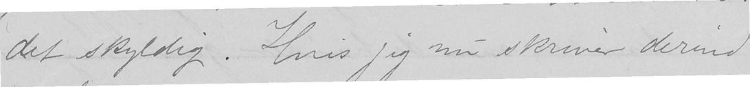det skyldig. Hvis jeg nu skriver derind
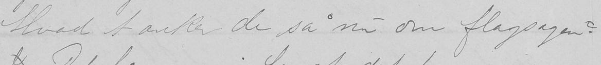Hvad tænker de så nu om flagsagen?
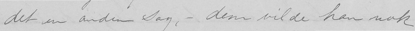det en anden sag, - dem vilde han nok
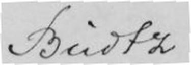Budtz
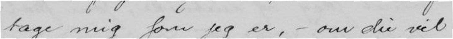tage mig som jeg er, - om du vil
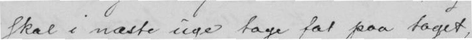skal i næste uge tage fat paa taget,
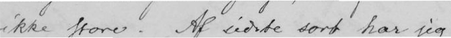ikke store. Af sidste sort har jeg
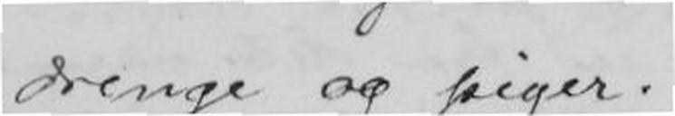drenge og piger.
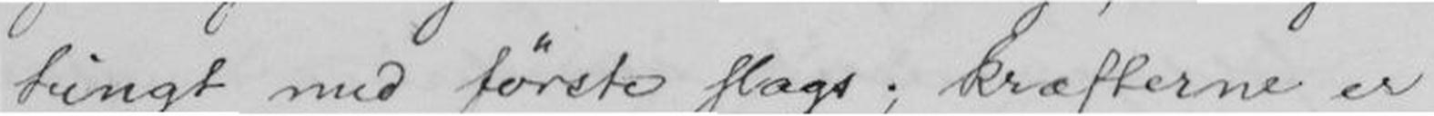tungt med første slags; kræfterne er
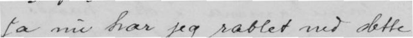Ja nu har jeg rablet ned dette
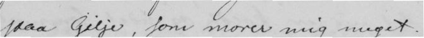paa Gilje, som morer mig meget.
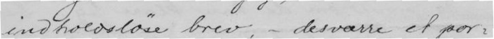indholdsløse brev. - desværre et por-
